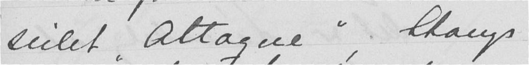seilet "Attaque", Stangs
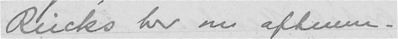Riecks her om aftenen.
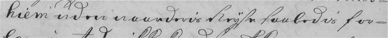hiem uden naar deris Reyse saaledis for-
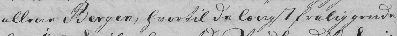allran Bergen, hvortil de længst fraliggende
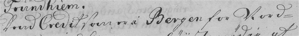Dend Credit som er i Bergen for Nord-
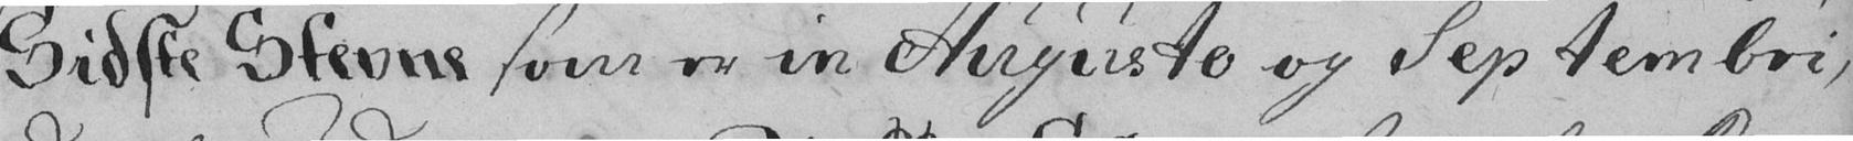Sidste Stevne som er in Augusto og Septembri,
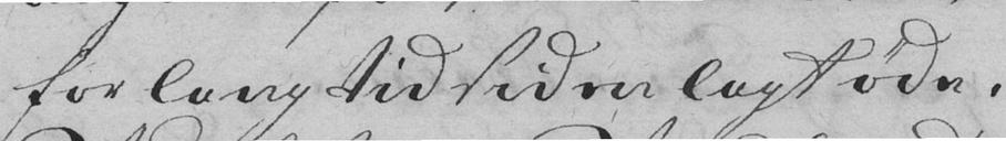for lang tid siden lagt øde.
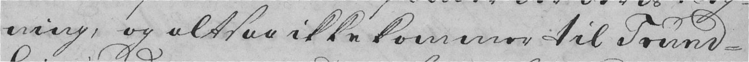ning, og altsaa ikke kommer til Trund-
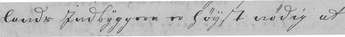lands Indbyggere er høyst nødig at
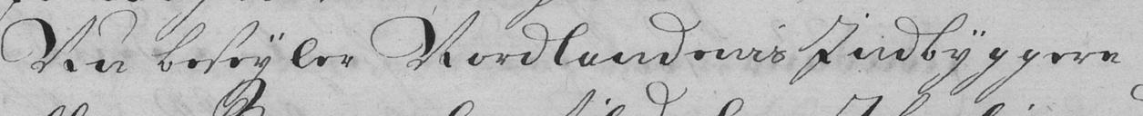Nu beseyler Nordlandenis Indbyggere
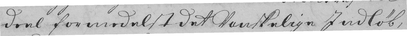deel formedelst det Vanskelige Indløb,
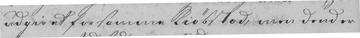udgivet for samme Kiøbstæd, men dend er
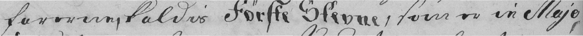farerne, kaldis Første Stevne, som er in Majo,
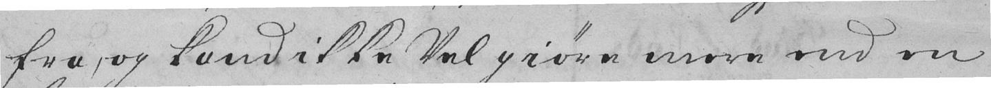fra, og kand ikke Velgiøre mere end en
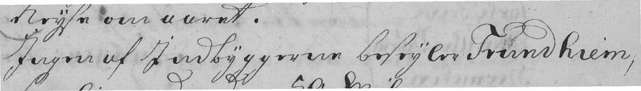Ingen af Indbyggerne beseyler Trundhiem,
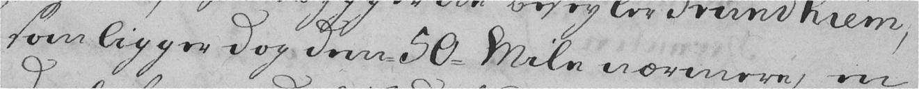som ligger dog dem 50 Mile nærmere, en
